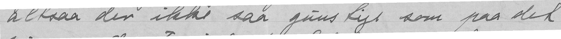altsaa der ikke saa gunstige som paa det
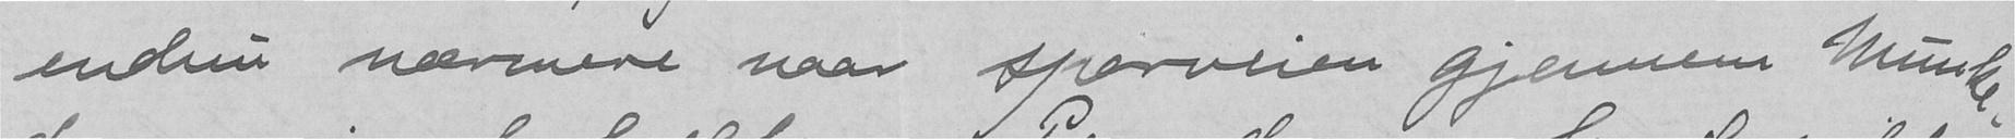endnu nærmere naar sporveien gjennem Munke-
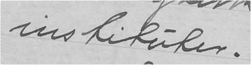instituter.
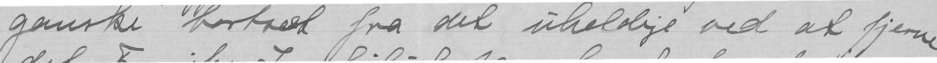ganske bortsæt fra det uheldige ved at fjerne
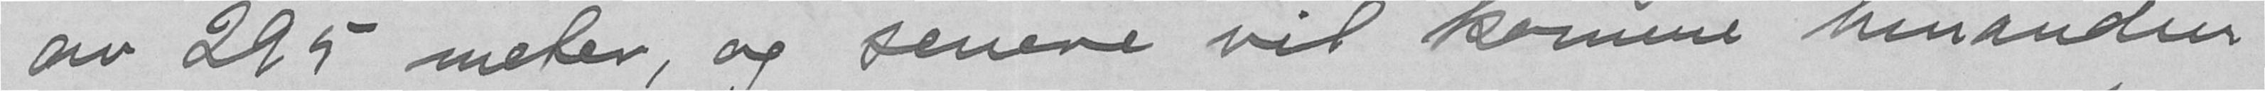av 295 meter, og senere vil komme hinanden
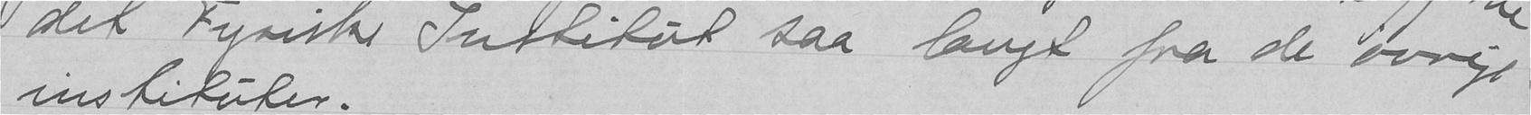det Fysiske Institut saa langt fra de øvrige
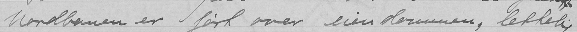Nordbanen er ført over eiendommen, lettelig
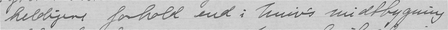heldigere fohold end i Univ's midtbygning
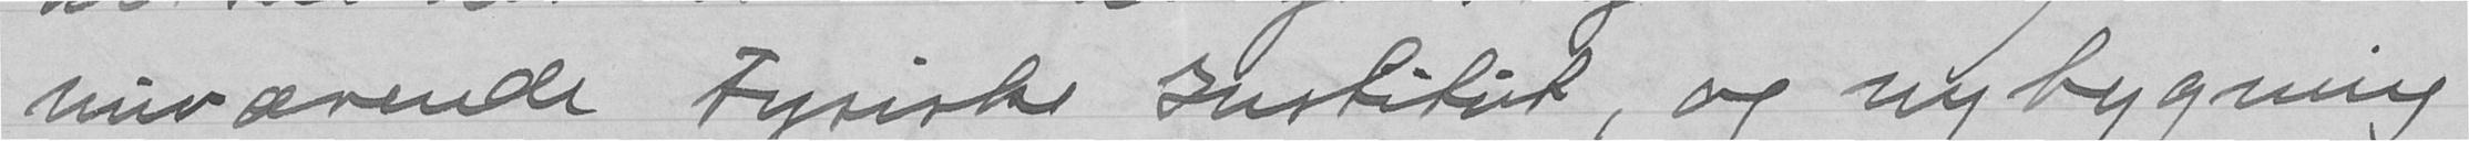nuværende Fysiske Institut, og nybygning
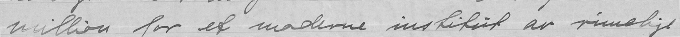million for et moderne institut av rimelige
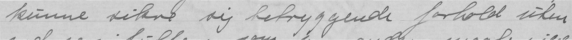kunne sikre sig betryggende forhold uten
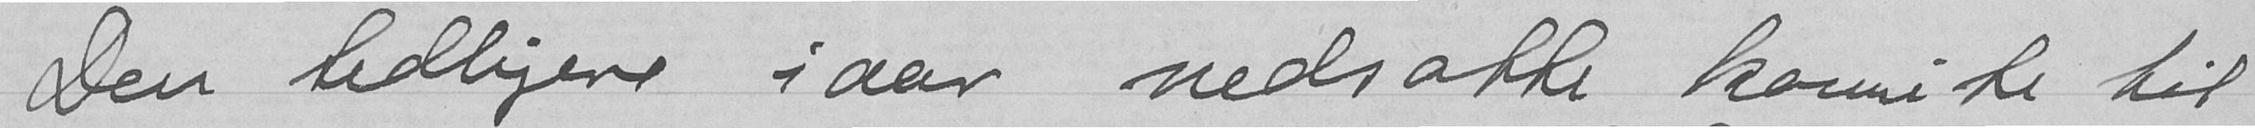Den tidligere i aar nedsatte komite til
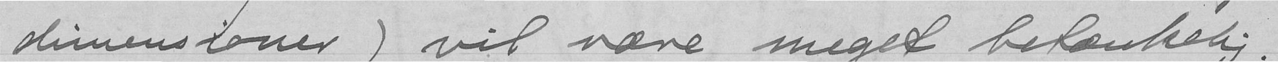dimensioner) vil være meget betænkelig.
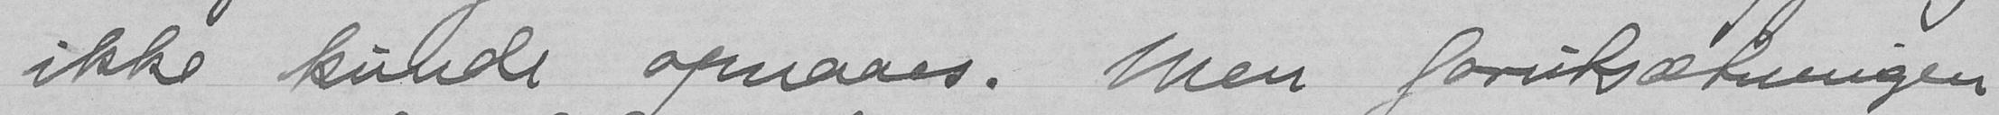ikke kunde opnaaes. Men forutsætningen
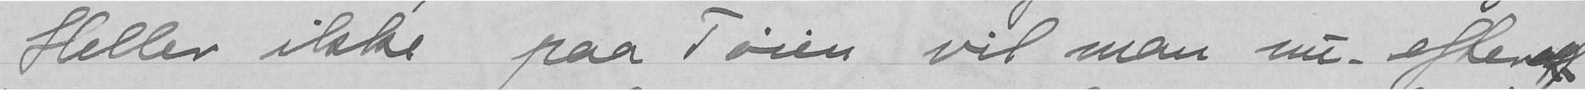Heller ikke paa Tøien vil man nu- efterat
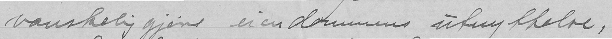vanskeliggjøre eiendommens utnyttelse,
